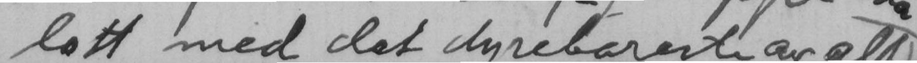lett med det dyrebareste av alt
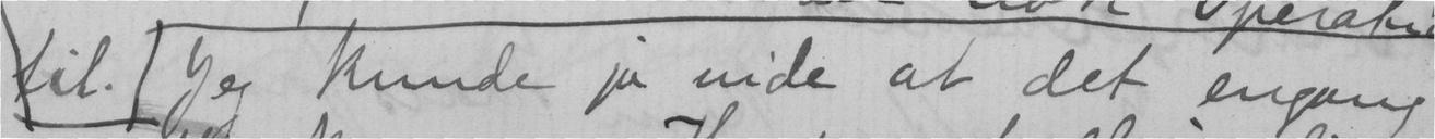til. Jeg kunde jo vide at det engang
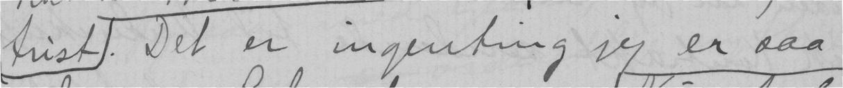trist. Det er ingenting jeg er saa
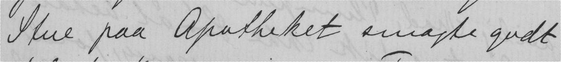Stue paa Apotheket smagte godt
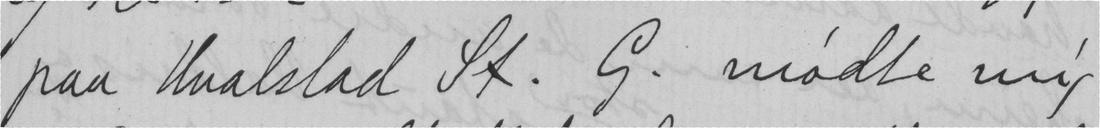paa Hvalstad St. G. mødte mig
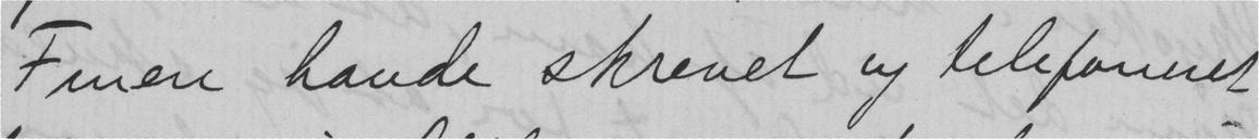Fruen havde skrevet og teleforeret
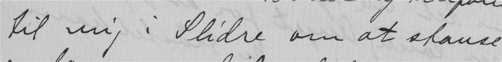til mig i Slidre om at stanse
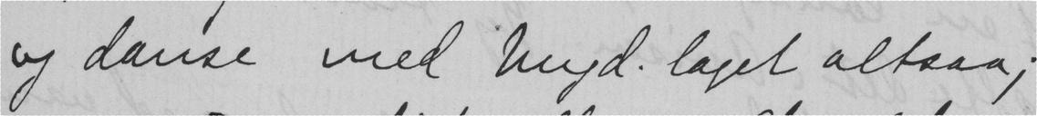og danse med ungd. laget altsaa;
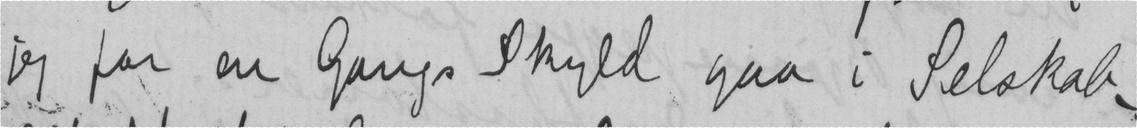jeg for en Gangs Skyld gaa i Selskab -
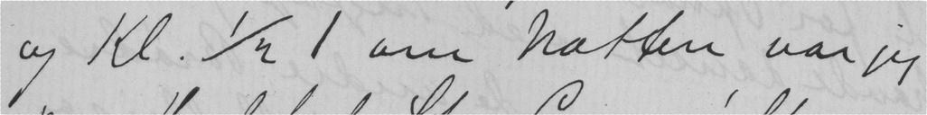og Kl. ½1 om natten var jeg
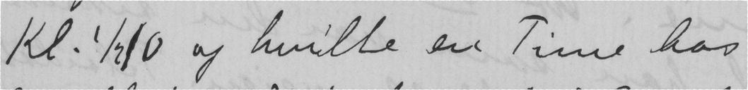Kl. 1/2 10 og hvilte en Time hos
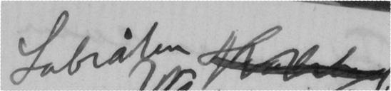Lobråten
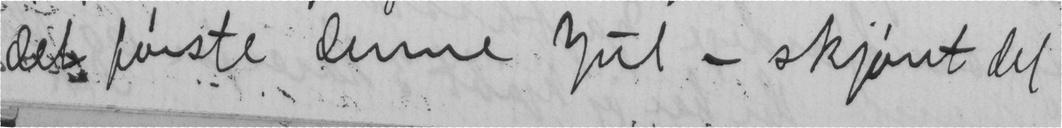det første denne Jul - skjønt det
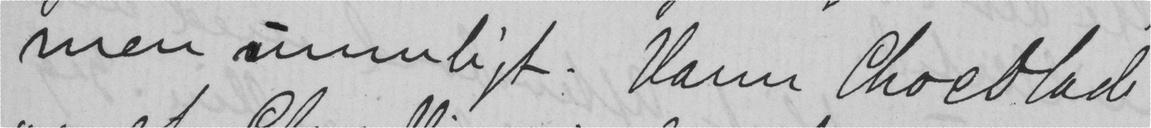men umuligt. Varm Chocolade
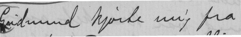Gudmind kjørte mig fra
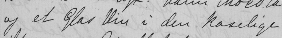og et Glas Vin i den koselige
